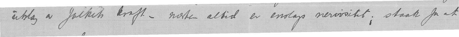utslag av folkets kraft, næsten altid er enslags nervøsitet; staak for at
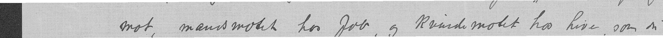i den, liv og romslighet. Vakrest av alt synes jeg er det præg av kraft og
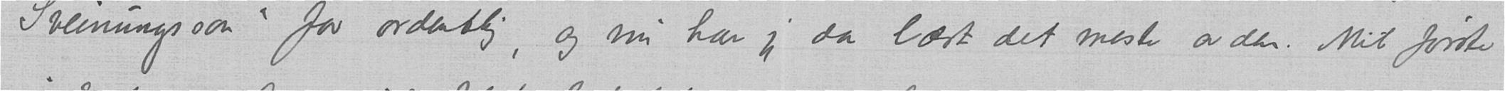Sveinungsson" for ordentlig, og nu har jeg da læst det meste av den. Mit første
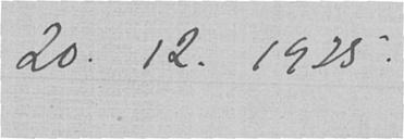20.12.1925.
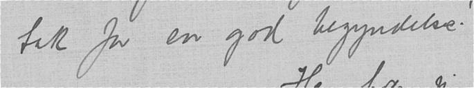tak for en god begyndelse!
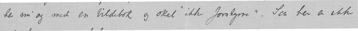her nu og med en bildebok og skal "ikke forstyrre". Saa her er ikke
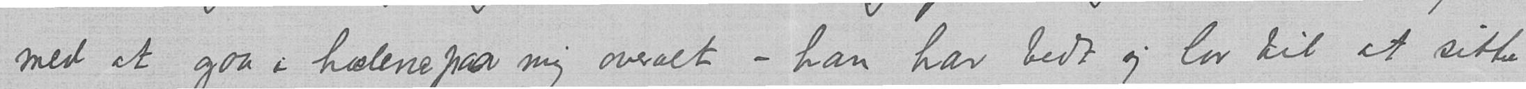med at gaa i hælene paa mig overalt – han har bedt sig lov til at sitte
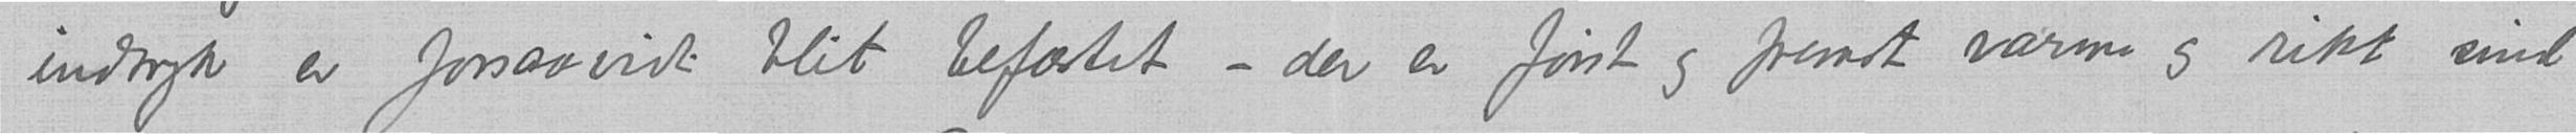indtryk er forsaavidt blit befæstet – der er først og fremst varme og rikt sind
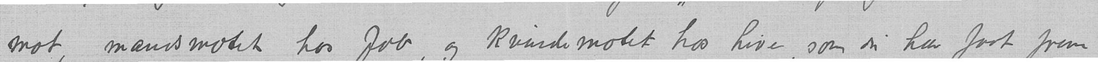mot, mandsmotet hos Job, og kvindemotet hos Live, som du har faat frem
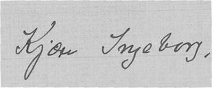Kjære Ingeborg,
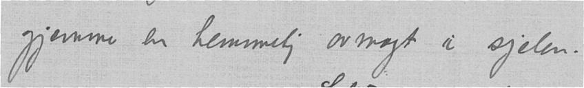gjemme en hemmelig avmagt i sjelen.
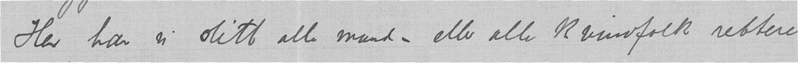Her har vi slitt alle mand - eller alle kvindfolk rettere
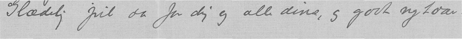Glædelig jul da for dig og alle dine, og godt nytaar.
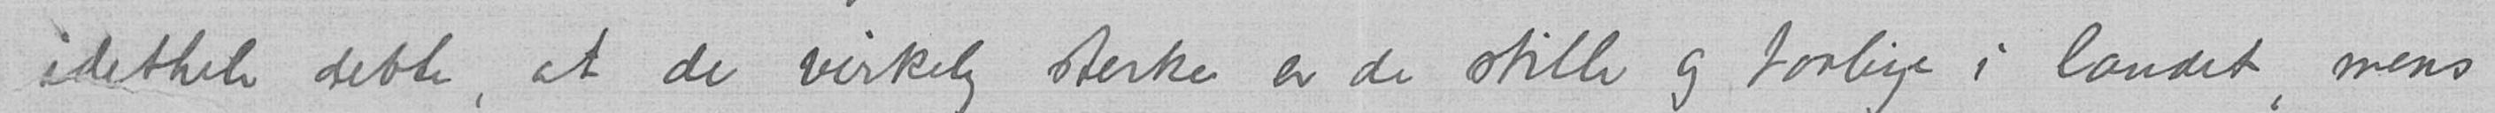- idethele dette, at de virkelig sterke er de stille og taalige i landet, mens
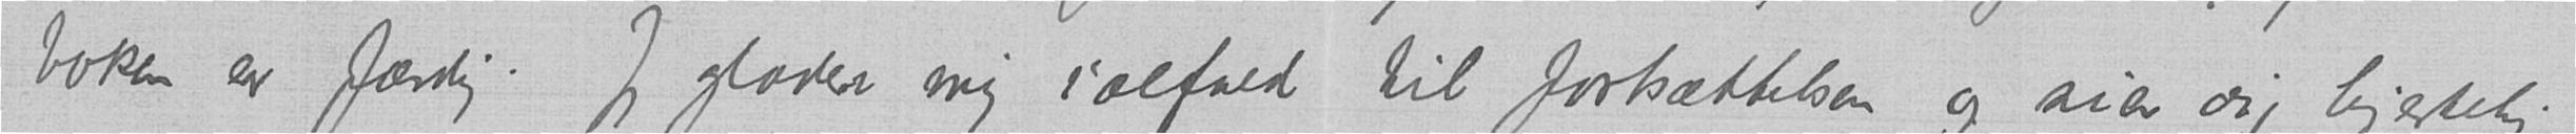boken er færdig. Jeg glæder mig ialfald til fortsættelsen og sier dig hjertelig
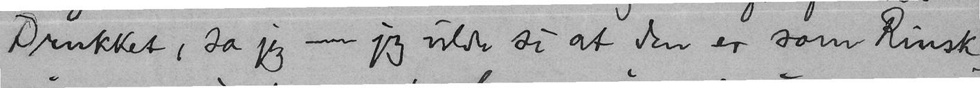Drukket, sa jeg - jeg vilde si at den er som Rinsk-
